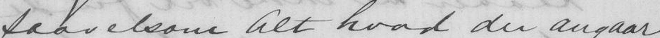faarelsom Alt hvad der angaar
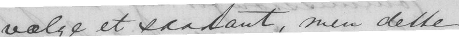vælge et saadant, men dette
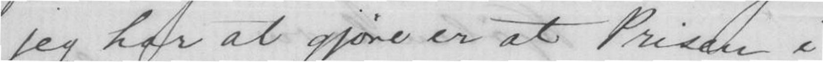jeg har at gjøre er at Prisen i
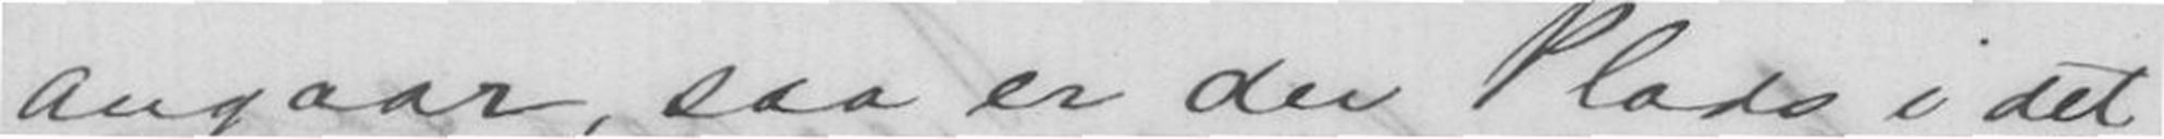angaar, saa er der Plads i det
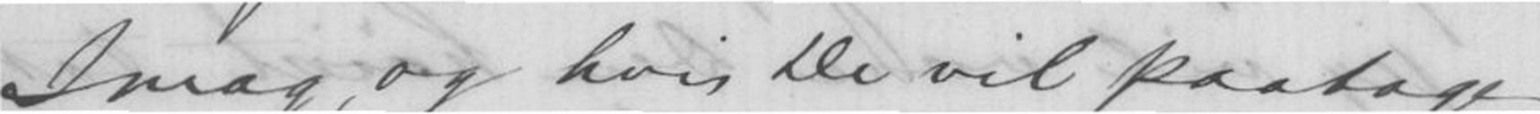Smag, og hvis De vil paatage
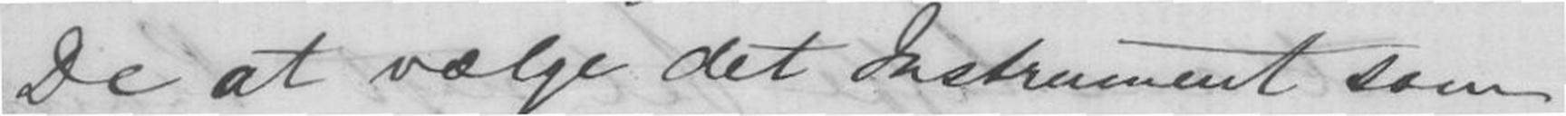De at vælge det Instrument som
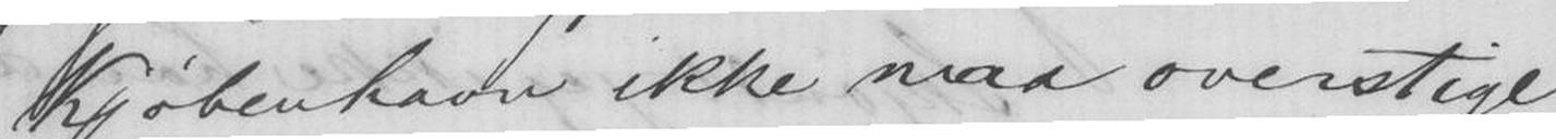Kjøbenhavn ikke maa overstige
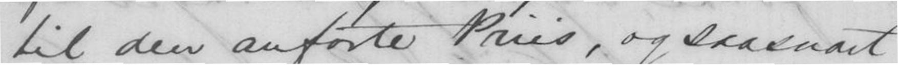til den anførte Priis, og saasnart
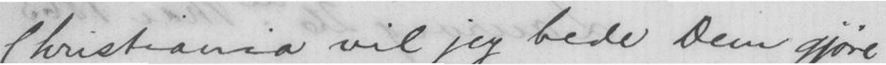Christiania vil jeg bede Dem gjøre
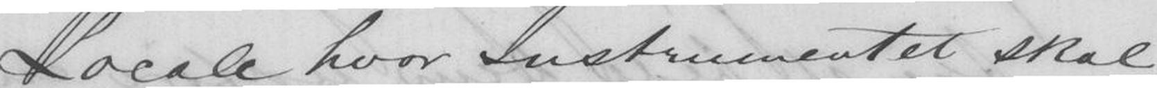Locale hvor Instrumentet skal
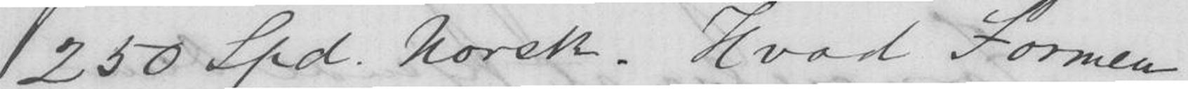250 Spd. Norsk. Hvad Formen
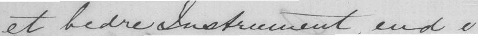et bedre Instrument, end i
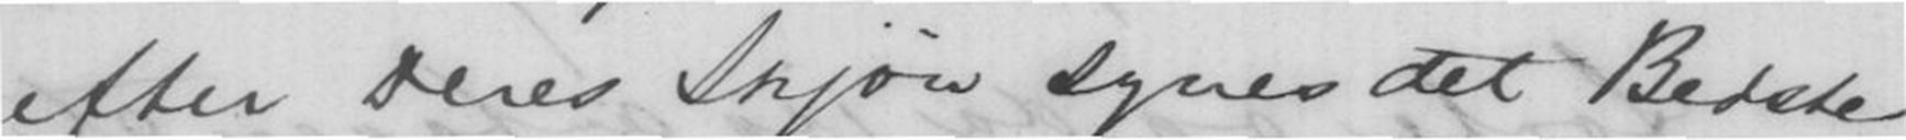efter Deres Skjøn synes det Bedste
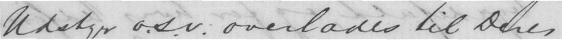Udstyr o.s.v. overlade til Deres
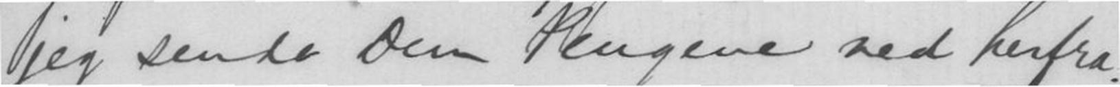jeg sende Den Pengene ned herfra
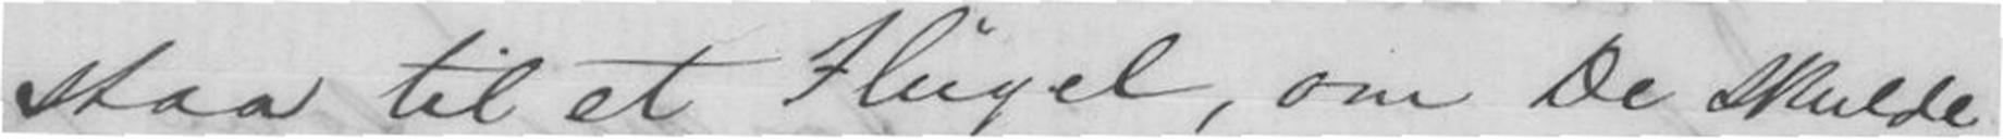staa til et Flugel, om De skulde
